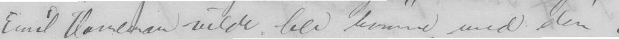Emil Horneman vilde her komme med den
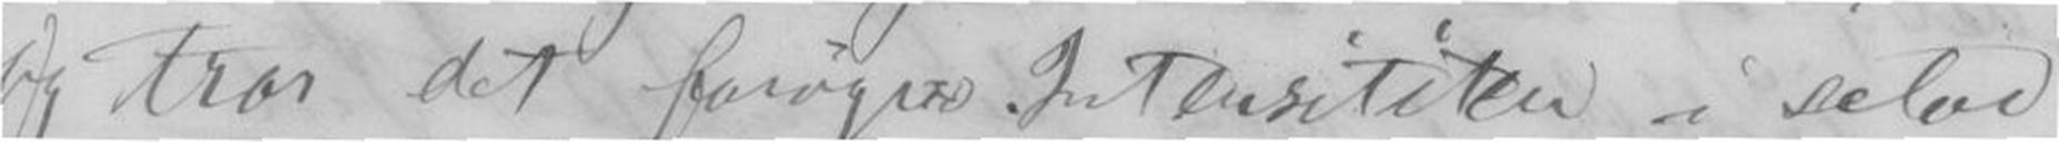jeg tror det forøger Intensiteten i selve
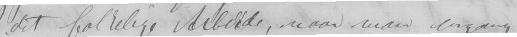det folkelige Arbeide, naar man engang
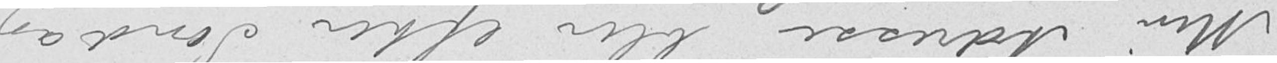Min Adresse blir efter Søndag
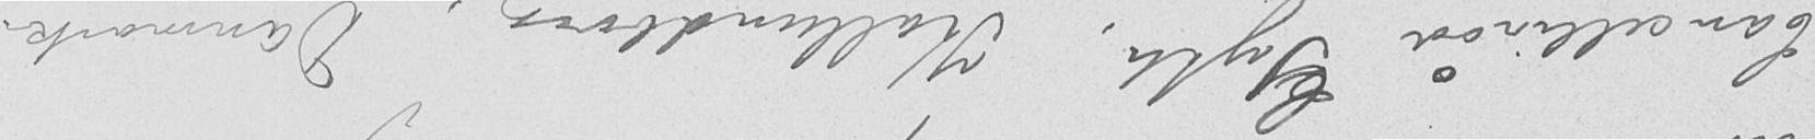Cancelliraad Gyth, Kallundborg, Danmark.
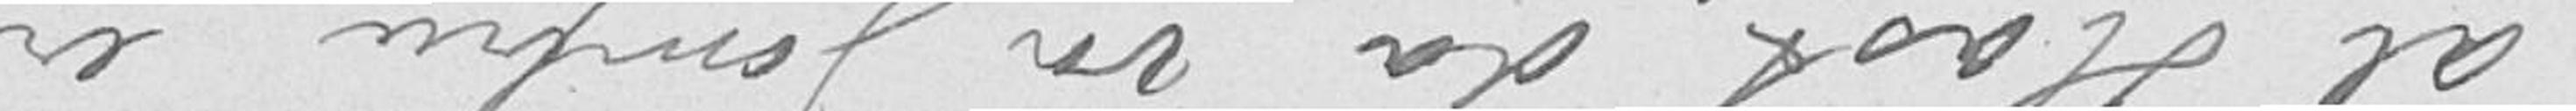al Hast, da vor Jomfru er
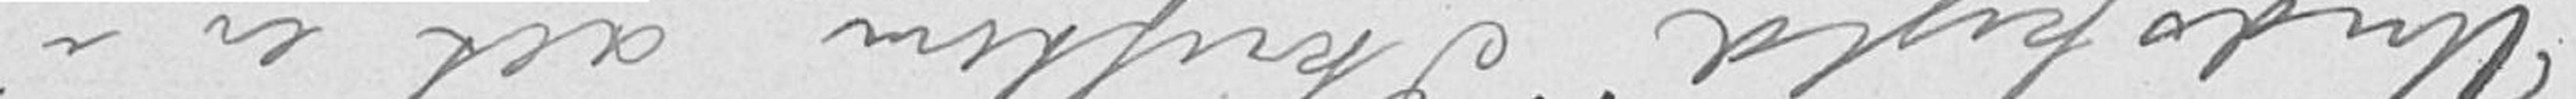Undskyld Skriften, alt er i
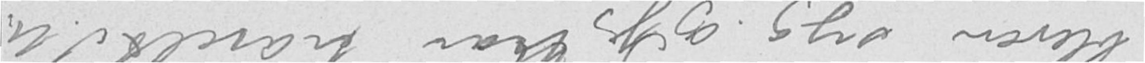bleven syg og jeg har travelt S.U
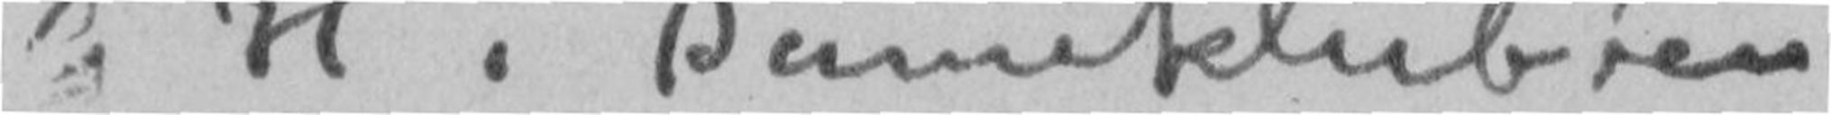G H i Dameklubben
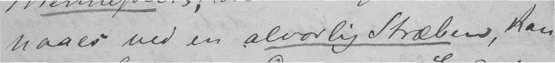naaes ved en alvorlig Stræben, kan
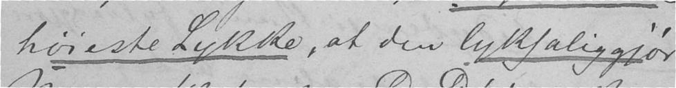høieste Lykke, at den lyksaliggjør
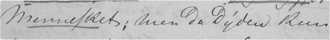Mennesket; men da Dyden kun
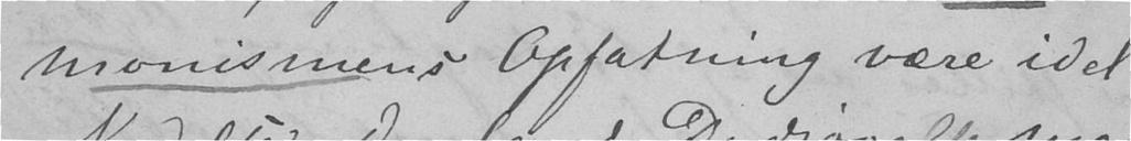monismens Opfatning være idel
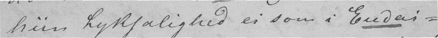hiin Lyksalighed ei som i Eudai-
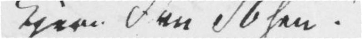Kjere Fru Ibsen!
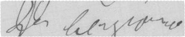Din hengivne
